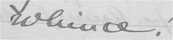Ewhiwce!
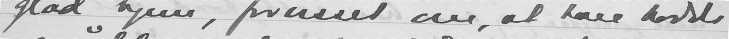glad hjem, forvisset om, at han hadde
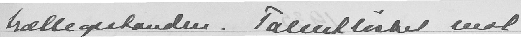trælleopstanden. Talentløshet mot
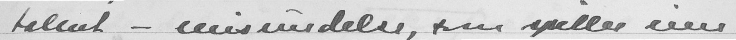talent - misundelse, som spiller inn
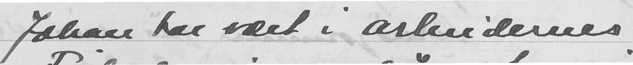Johan har vært i arbeidernes
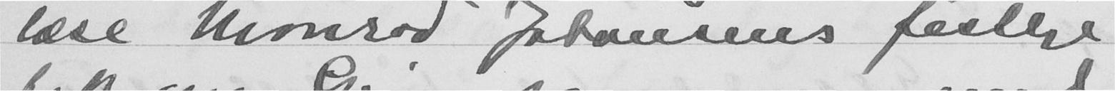læse Monrad Johansens festlige
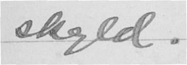skyld.
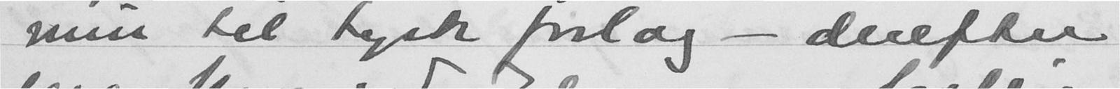min til tysk forlag - derefter
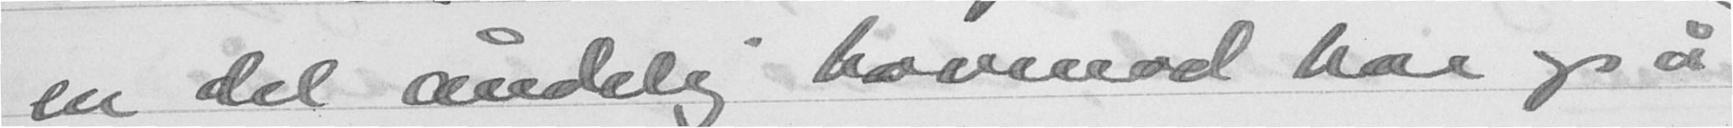en del åndelig hovmod har på
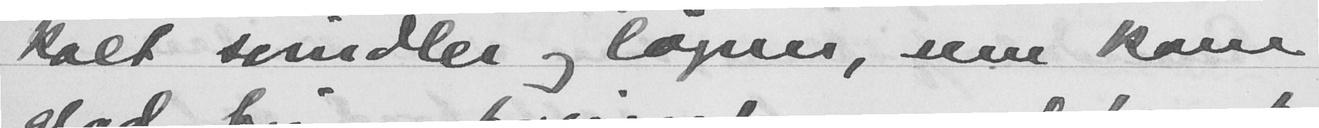kalt svindler og løgner, men kom
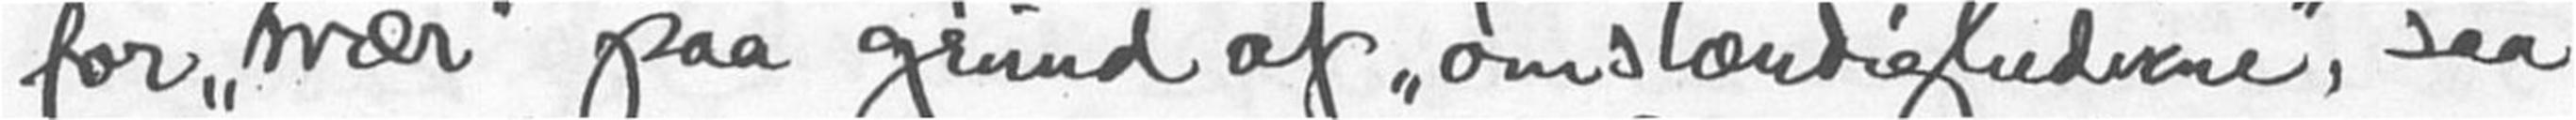for "svær" paa grund af "omstændighederne", saa
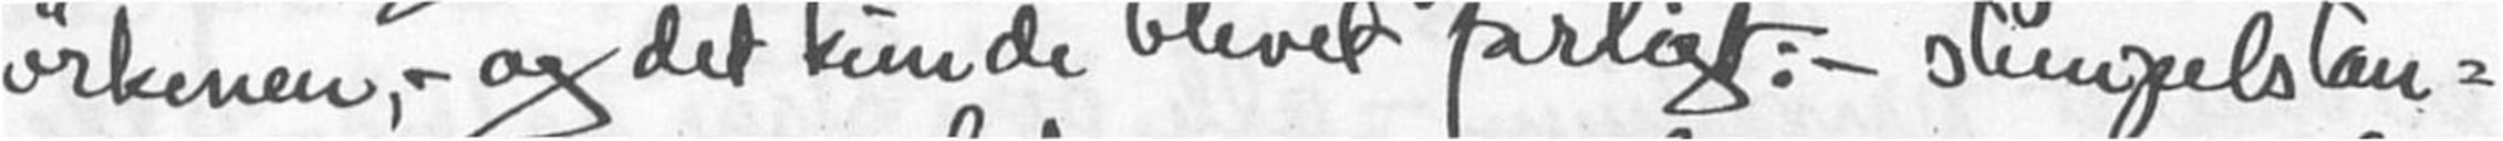ørkenen, -og det kunde blevet farligt; - stempelstan-
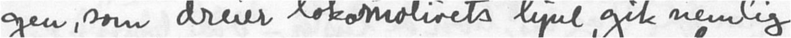gen, som dreier lokomotivets hjul gik nemlig
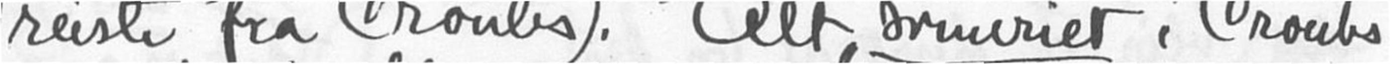reiste fra Croubs). Alt "svineriet" i Croubs
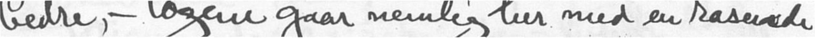bedre, - togene gaar nemlig her med en rasende
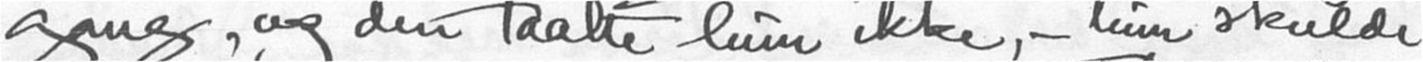gang, og den taalte hun ikke, - hun skulde
